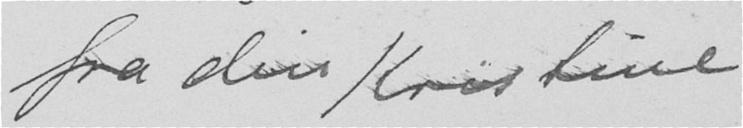fra din Kristine.
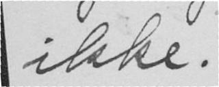ikke.
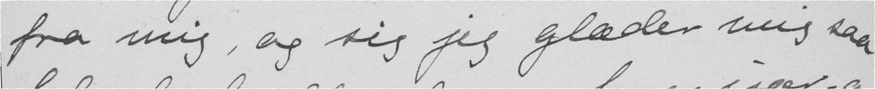fra mig, og sig jeg glæder mig saa
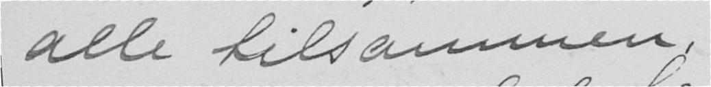alle tilsammen, -
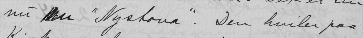nu  "Nystova". Den hviler paa
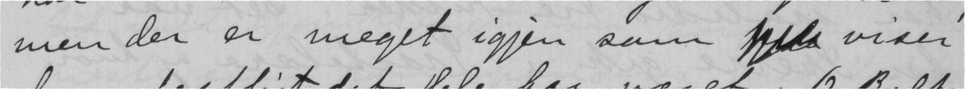men der er meget igjen som  viser
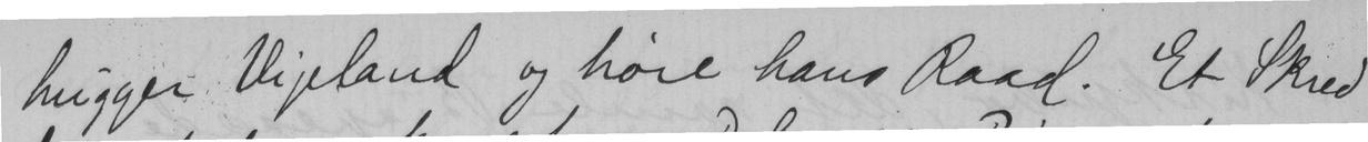hugger Vigeland og høre hans Raad. Et Skred
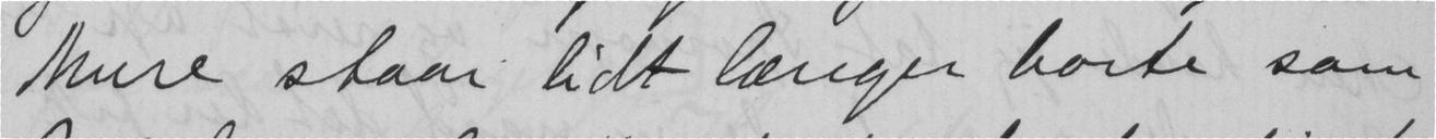Mure staar lidt længer borte som
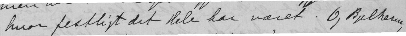hvor festligt det Hele har været. Og Bjelkerne,
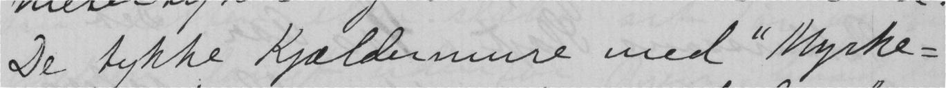De tykke Kjældermure med "Myrke-
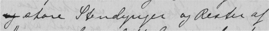store Stendynger og Rester af
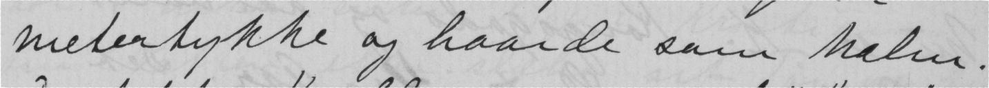metertykke og haarde som Malm.
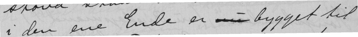i den ene Ende er  bygget til
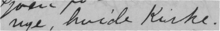nye, hvide Kirke.
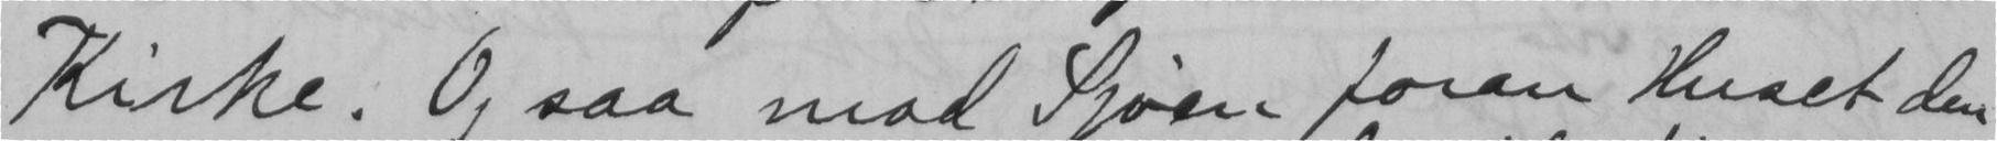Kirke. Og saa mod Sjøen foran Huset den
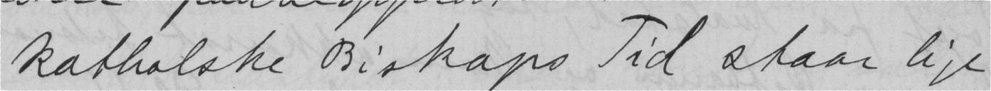katholske Biskops Tid staar lige
